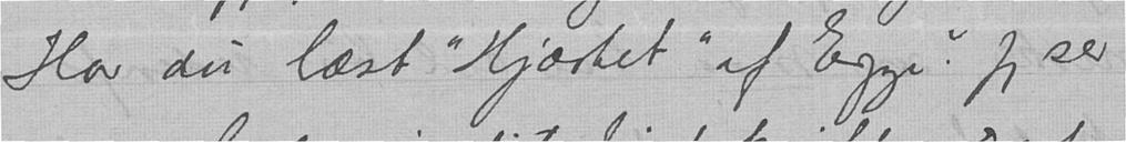Har du læst "Hjærtet" af Egge. Jeg ser
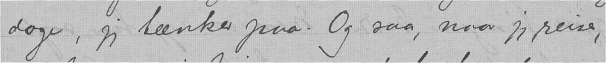dage, jeg tænker paa. Og saa, naar jeg reiser,
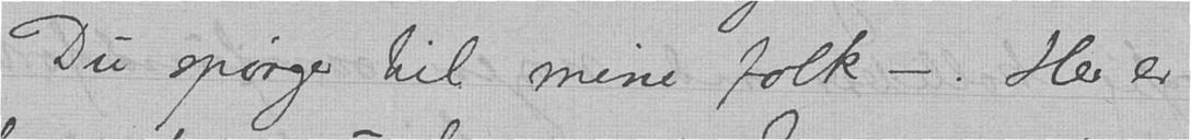Du spørger til mine folk -. Her er
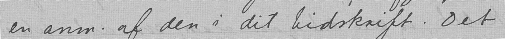en anm. af den i dit tidskrift. Det
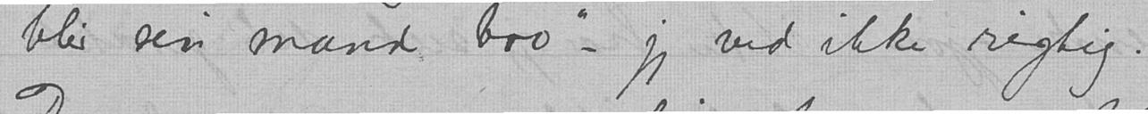blir sin mand tro" - jeg ved ikke rigtig.
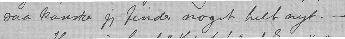saa kanske jeg finder noget helt nyt. -
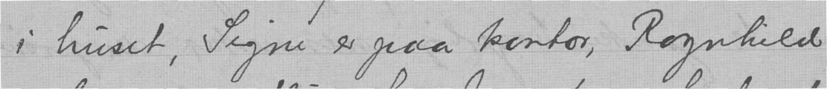i huset, Signe er paa kontor, Ragnhild
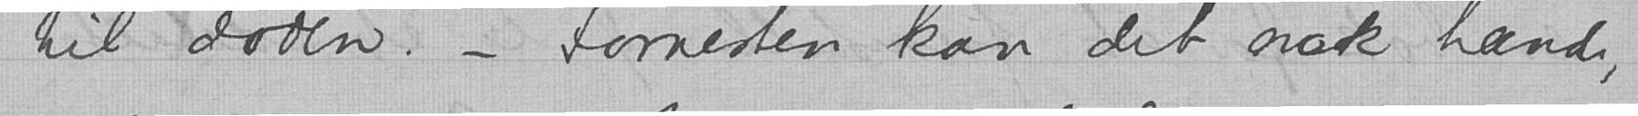til døden. - Forresten kan det nok hænde,
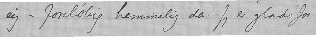sig - foreløblig hemmelig da. Jeg er glad for
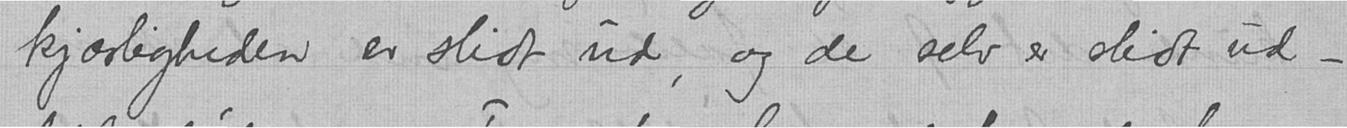kjærligheden er slidt ud, og de selv er slidt ud -
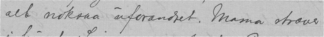alt noksaa uforandret. Mama stræver
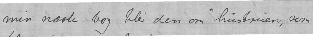min næste bog blir den om "hustruen, som
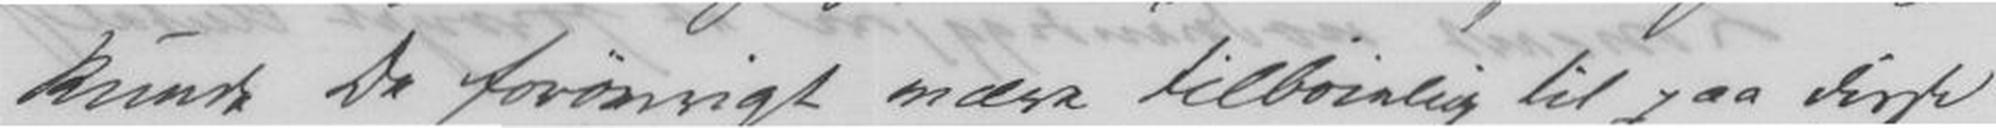kunde De forøvrigt være tilbøielig til paa disse
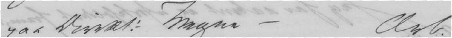paa Direkt: Vegne - Ærb.
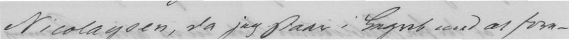Nicolaysen, da jeg staar i Begreb med at fore-
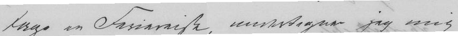tage en Feriereise, undertegner jeg mig
